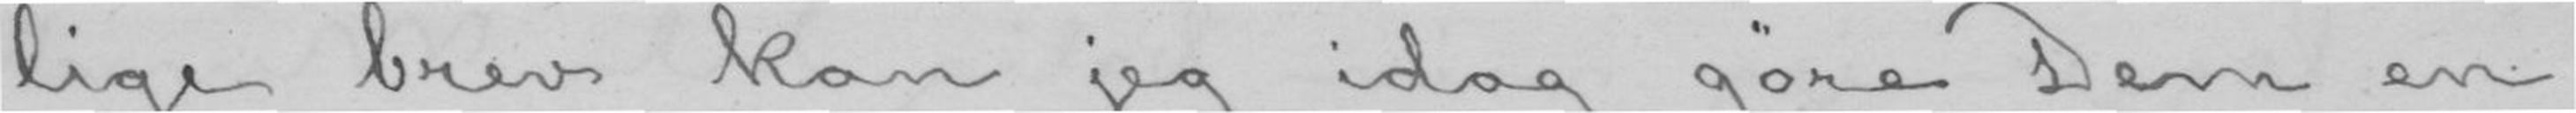lige brev kan jeg idag gøre Dem en
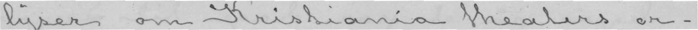lyser om Kristiania theaters or-
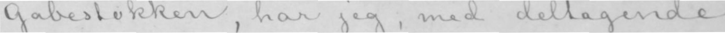Gabestokken, har jeg, med deltagende
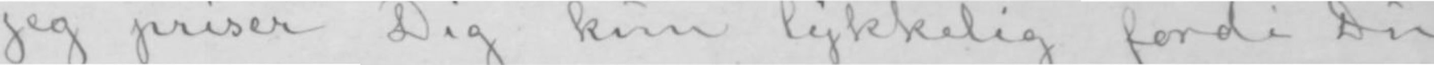jeg priser Dig kun lykkelig fordi Du
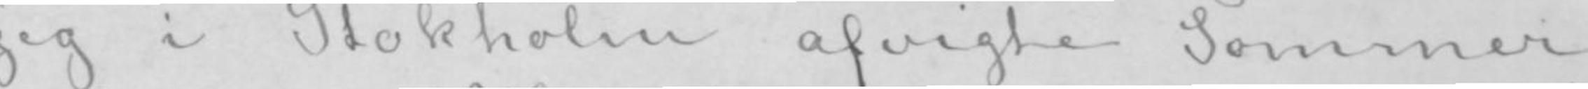jeg i Stokholm afvigte Sommer
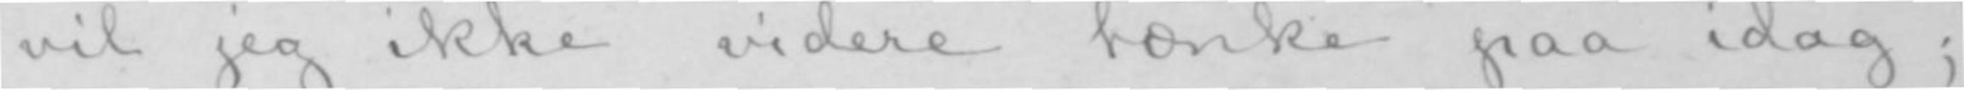vil jeg ikke videre tænke paa idag;
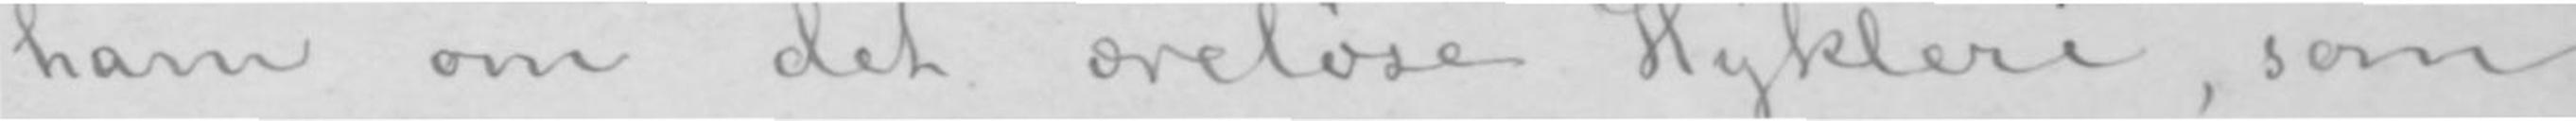ham om det æreløse Hykleri, som
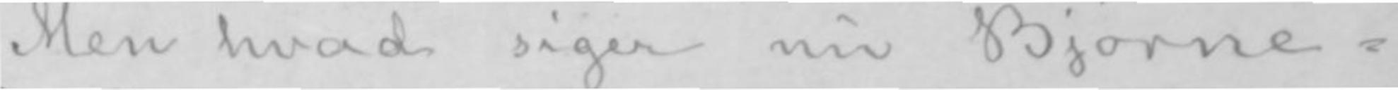Men hvad siger nu Bjørne-
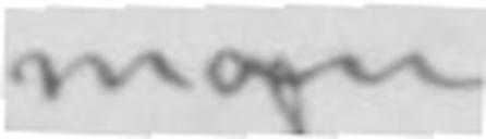man
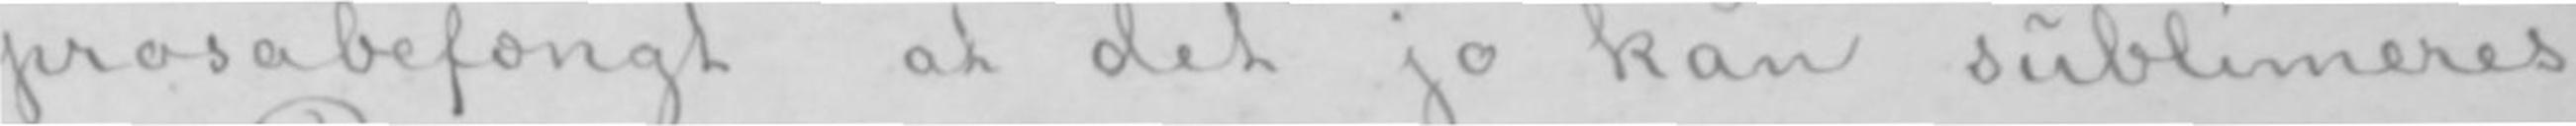prosabefængt at det jo kan sublimeres
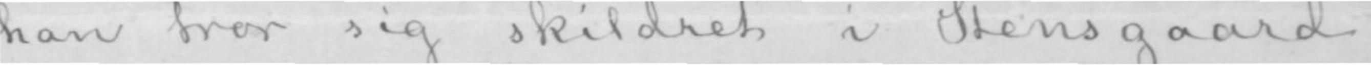han tror sig skildret i Stensgaard.
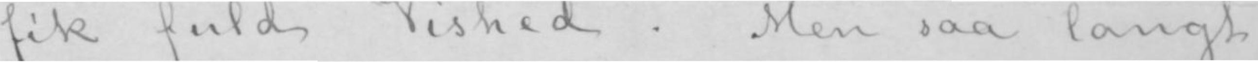fik fuld Vished. Men saa langt
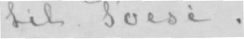til Poesi.
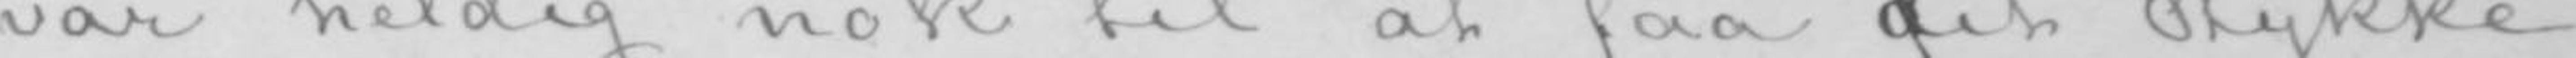var heldig nok til at faa ditf Stykke
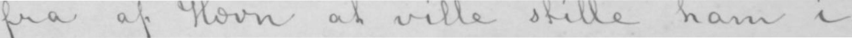fra af Hævn at ville stille ham i
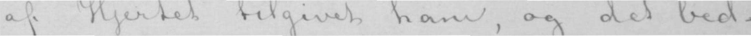af Hjertet tilgivet ham, og det bed-
